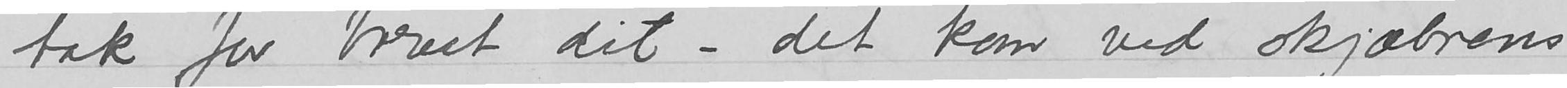tak for brevet dit – det kom ved skjæbnens
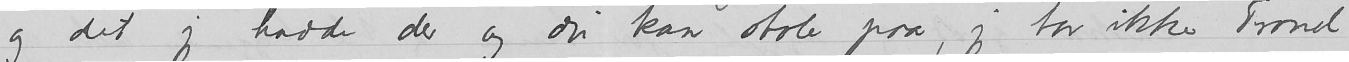og det jeg hadde der og du kan stole paa, jeg tar ikke Trond
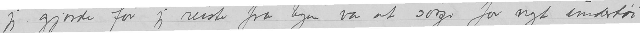jeg gjorde før jeg reiste fra byen var at sørge for nyt undertøi
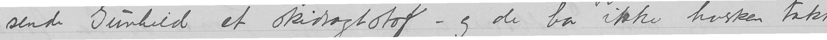sende Gunhild et skidragtstof – og de har ikke hverken takket
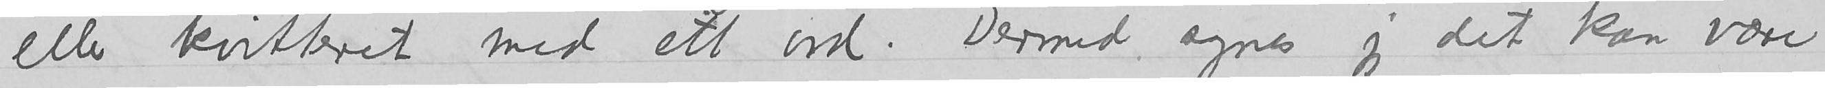eller kvittert med ett ord. Dermed synes jeg det kan være
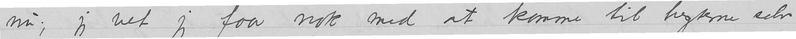nu; jeg vet jeg faar nok med at komme til hegterne selv
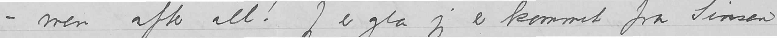–men after all! Jeg er gla jeg er kommet fra Sinsen
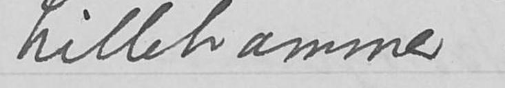Lillehammer.
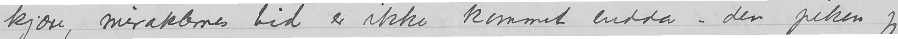kjære, miraklernes tid er ikke kommet endda – den piken jeg
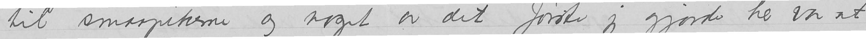til smaapikerne og noget at det første jeg gjorde her var at
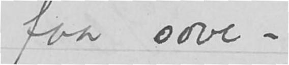faa sove –.
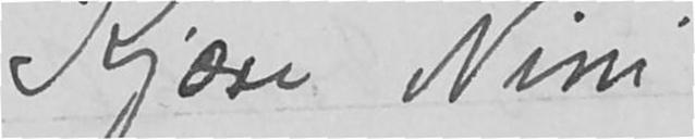Kjære Nini,
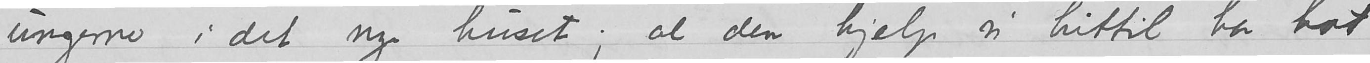ungerne i det nye huset; al den hjelp vi hittil har hat
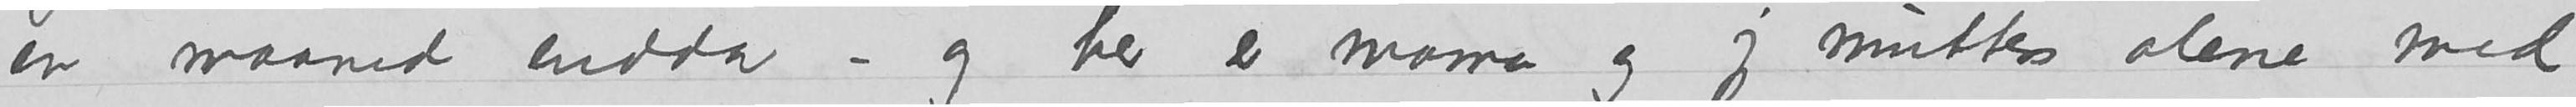en maaned endda – og her er mama og jeg mutters alene med
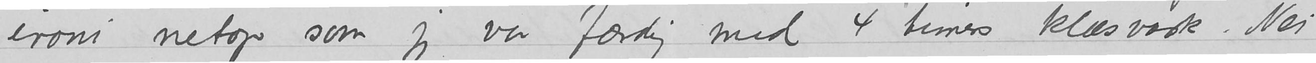ironi netop som jeg var færdig med 4 timers klæsvask. Nei
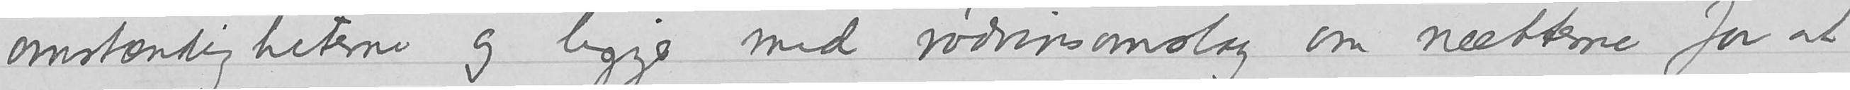omstændigheterne og ligger med rødvinsomslag om nætterne for at
